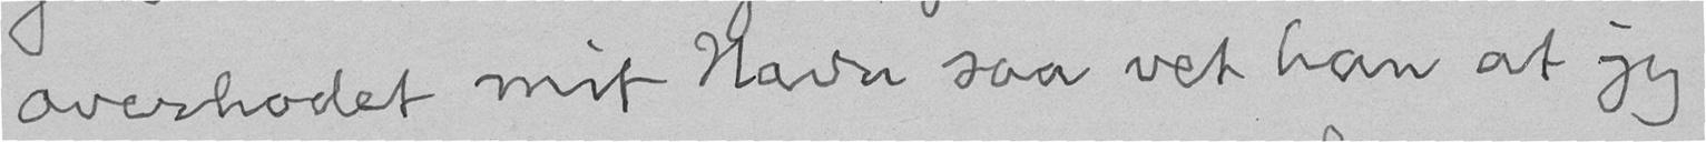overhodet mit Navn saa vet han at jeg
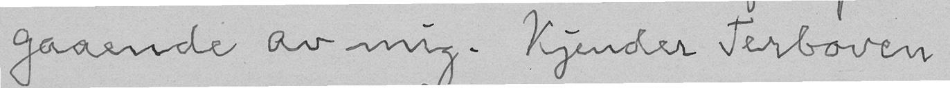gaaende av mig. Kjender Terboven
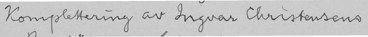Komplettering av Ingvar Christensens
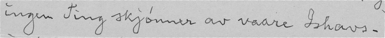ingen Ting skjønner av vaare Ishavs-
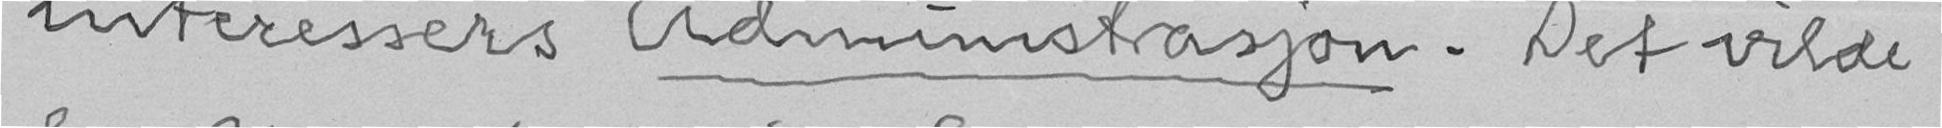interessers Administrasjon. Det vilde
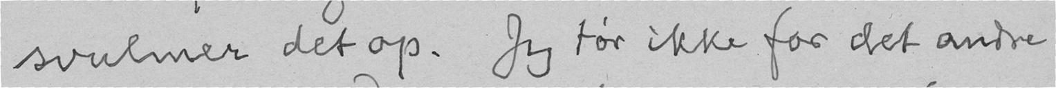svulmer det op. Jeg tør ikke for det andre
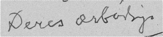Deres ærbødige
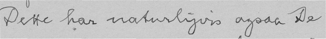Dette har naturligvis ogsaa De
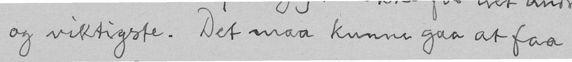og viktigste. Det maa kunne gaa at faa
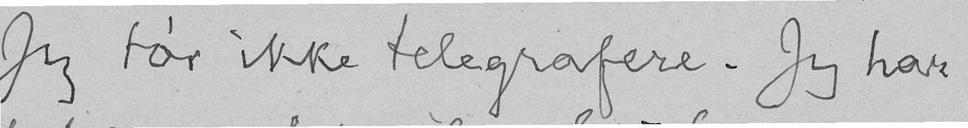Jeg tør ikke telegrafere. Jeg har
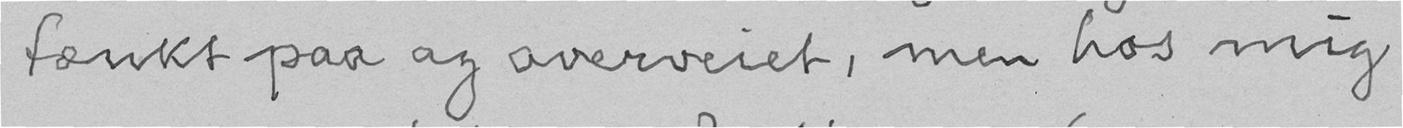tænkt paa og overveiet, men hos mig
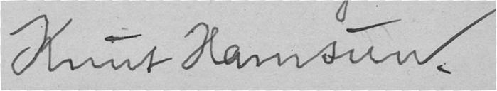Knut Hamsun.
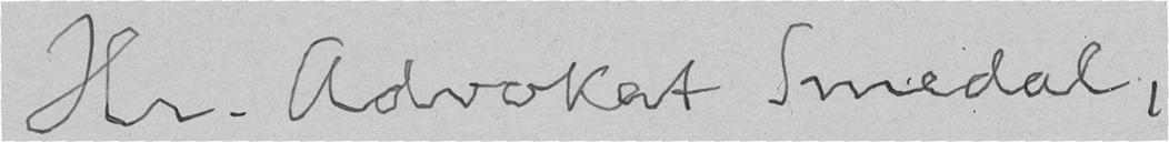Hr. Advokat Smedal,
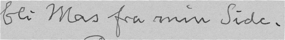bli Mas fra min Side.
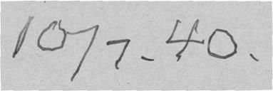10/7. 40.
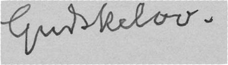Gudskelov.
